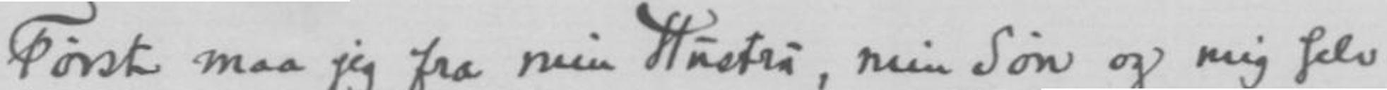Først maa jeg fra min Hustru, min Søn og mig selv
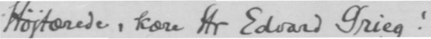Højtærede, kære Hr Edvard Grieg!
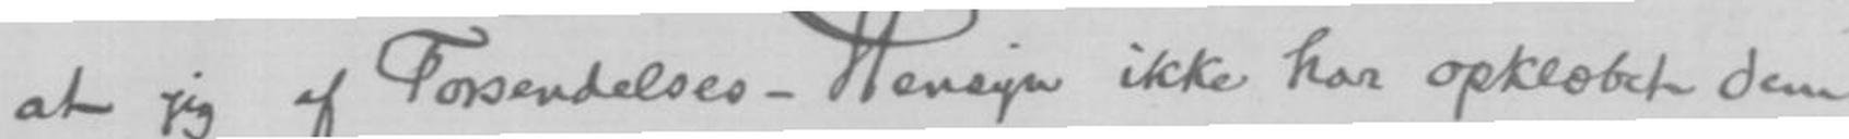at jeg af Forsendelses - Hensyn ikke har opklæbete dem
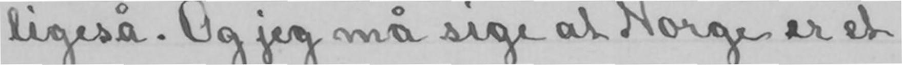ligeså. Og jeg må sige at Norge er et
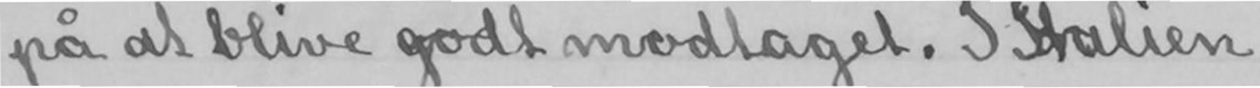på at blive godt modtaget. I Italien
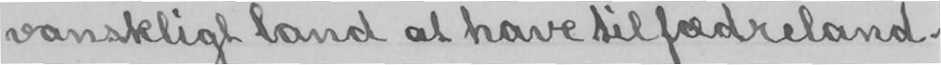vanskligt land at have til fædreland.
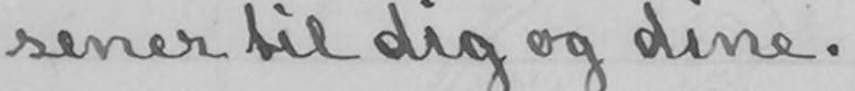sener til dig og dine.
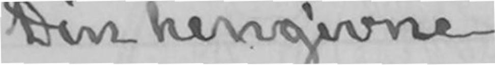Din hengivne
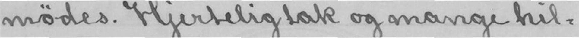mødes. Hjertelig tak og mange hil-
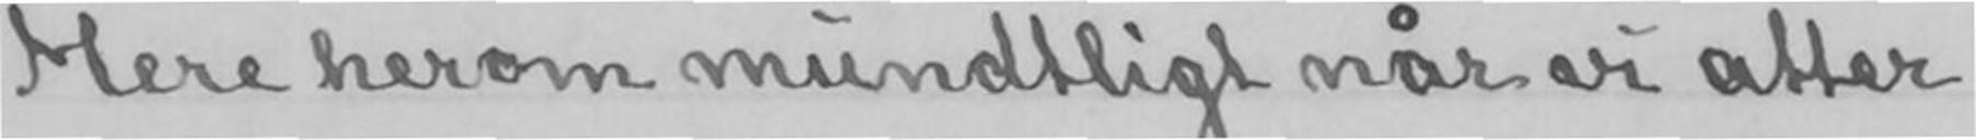Mere herom mundtligt når vi atter
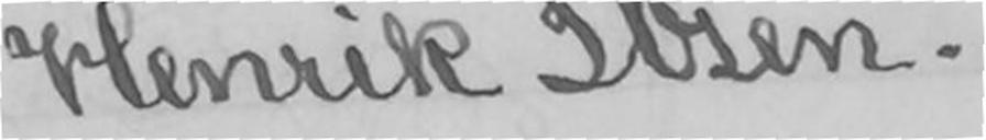Henrik Ibsen.
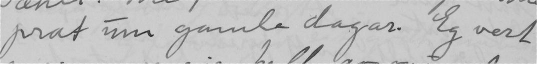prat um gamle dagar. Eg vert
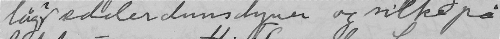låg i edderdunsdyner og silke på
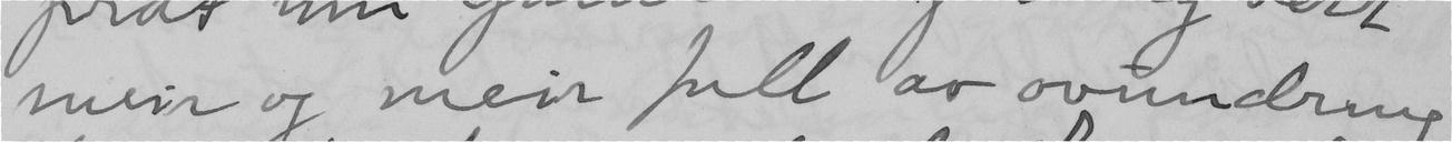meir og meir full av ovundring
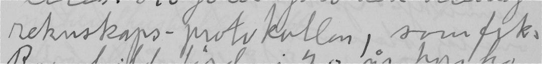reknskaps-protokollen, som frk.
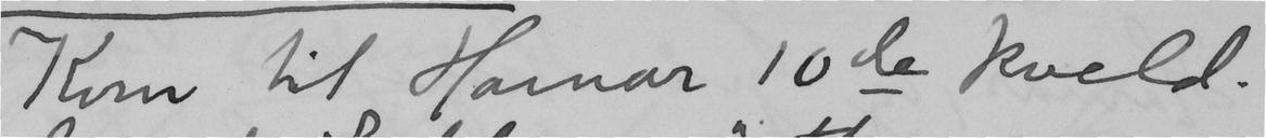Kom til Hamar 10de kveld.
